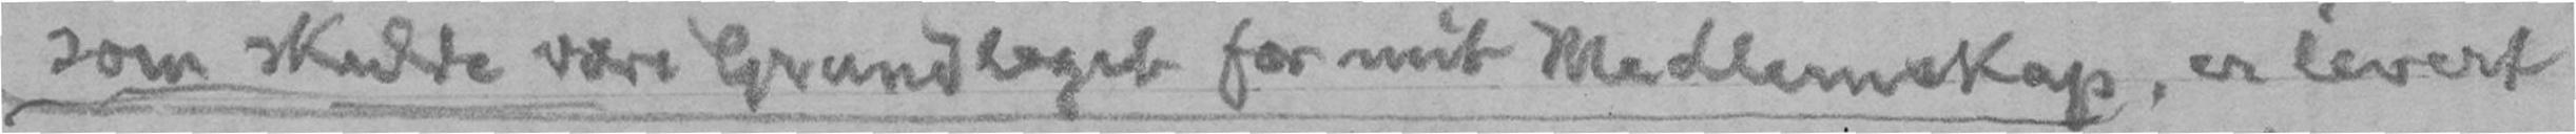som skulde være Grundlaget for mit Medlemskap, er levert
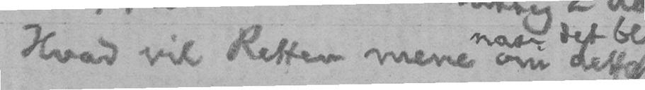Hvad vil Retten mene om dette
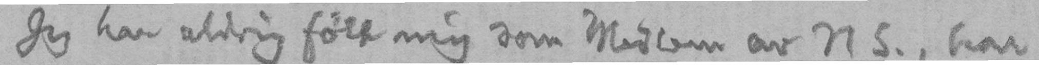Jeg har aldrig følt mig som Medlem av NS., har
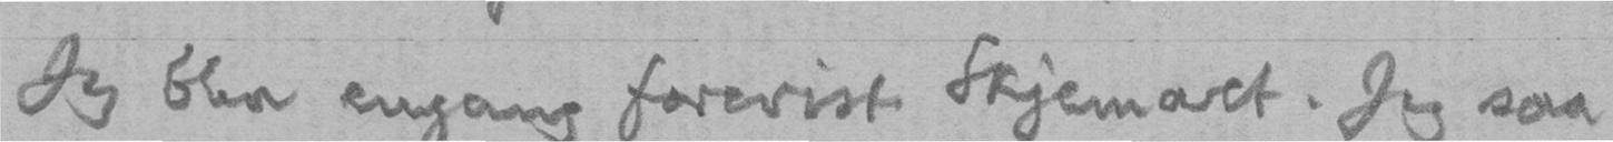Jeg blev engang forevist Skjemaet. Jeg saa
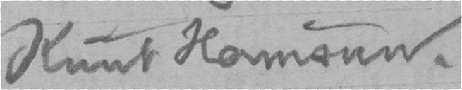Knut Hamsun.
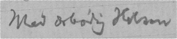Med ærbødig Hilsen
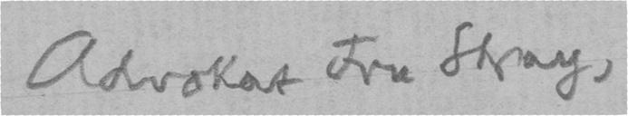Advokat Fru Stray,
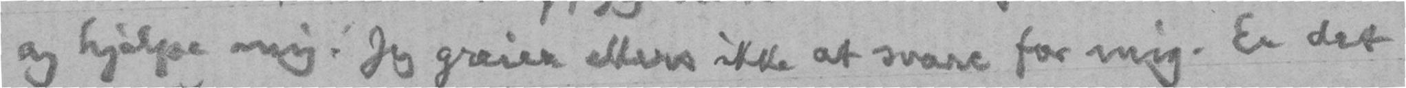og hjelpe mig! Jeg greier ellers ikke at svare for mig. Er det
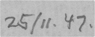25/11. 47.
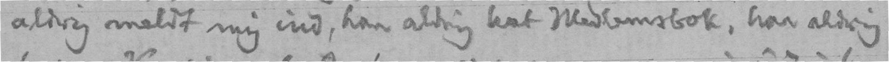aldrig meldt mig ind, har aldrig hat Medlemsbok, har aldrig
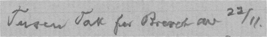Tusen Tak for Brevet av 22/11.
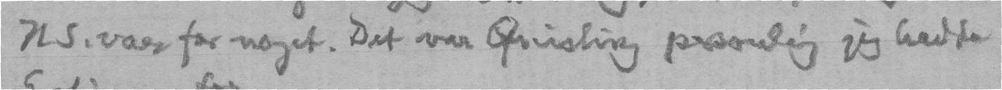NS. var for noget. Det var Quisling personlig jeg hadde
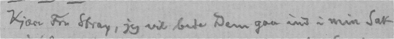Kjære Fru Stray, jeg vil bede Dem gaa ind i min Sak
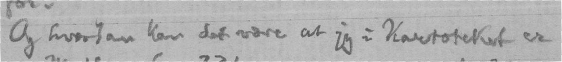Og hvordan kan det være at jeg i Kartoteket er
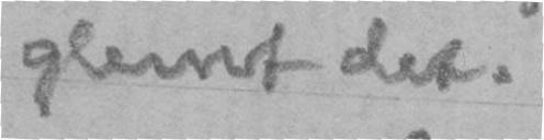glemt det.
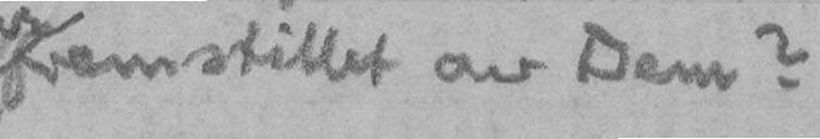fremstillet av Dem?
